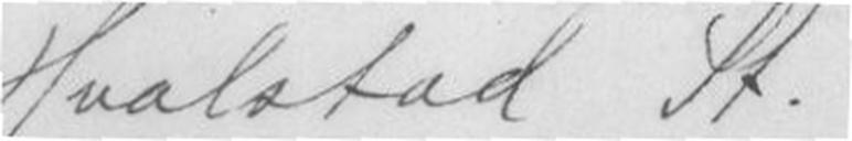Hvalstad St.
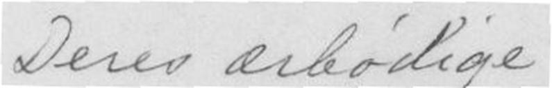Deres ærbødige
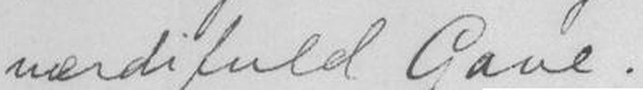verdifuld Gave.
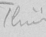Thiis
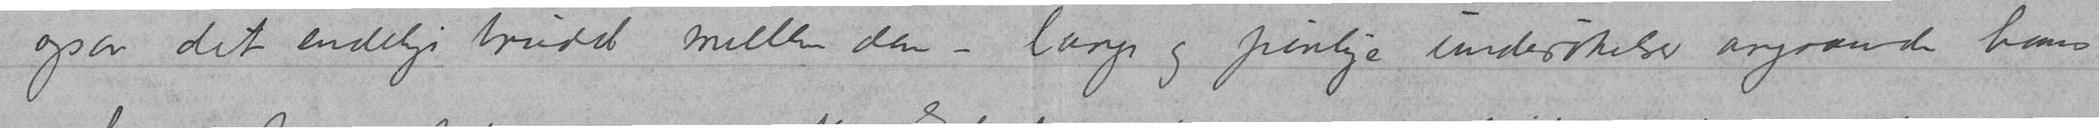ogsaa det endelige brudd mellem dem – lange og pinlige undersøkelser angaaende hans
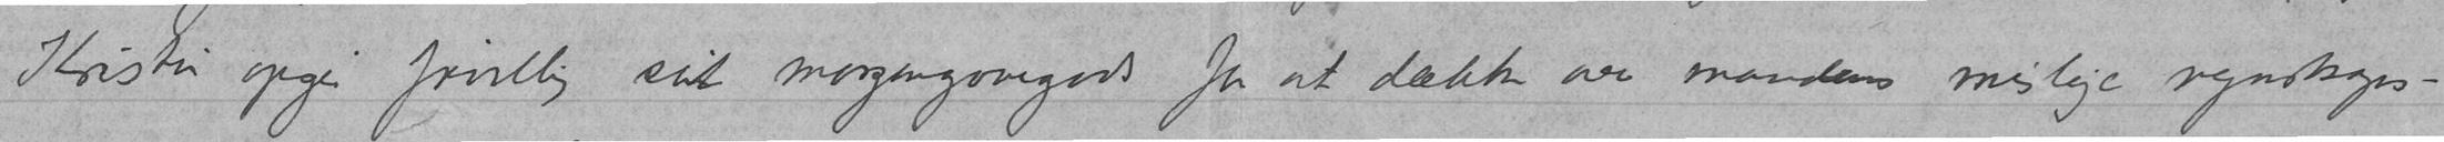Kristin opgir frivllig sit morgengavegods for at dække over mandens mislige regnskaps-
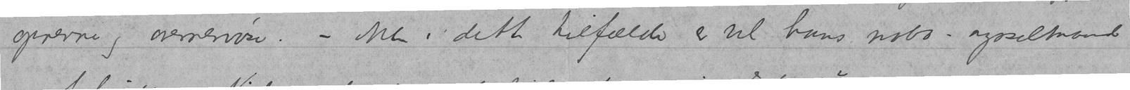oprevne og overnervøse. – Men i dette tilfælde er vel hans nabo-sysselmand
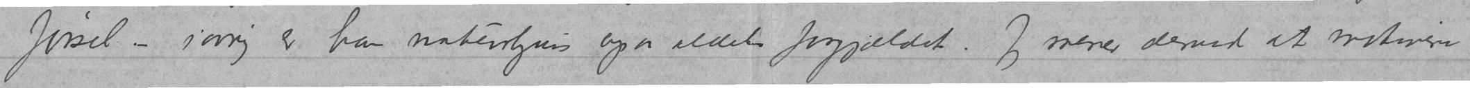førsel – iøvrig er han naturligvis ogsaa aldeles forgjældet. Jeg mener derved at motivere
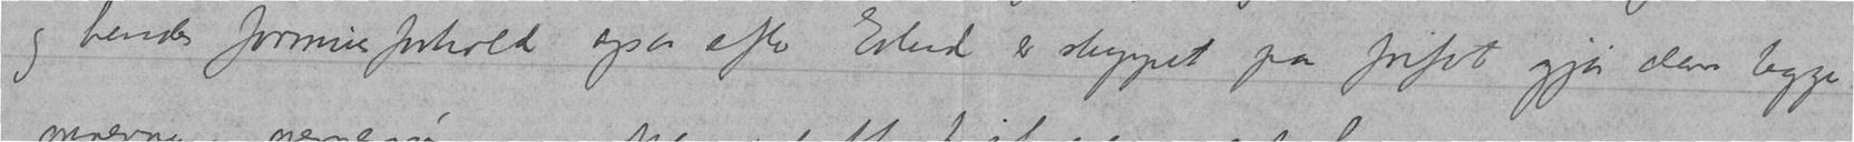og hendes formuesforhold ogsaa efter Erlend er sluppet paa frifot gjør dem begge
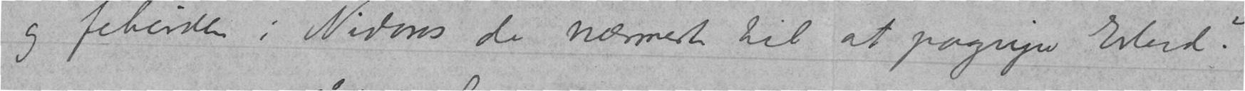og fehirden i Nidaros de nærmeste til at pågripe Erlend?
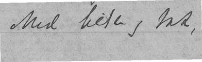Med hilsen og tak,
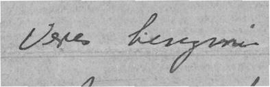Deres hengivne
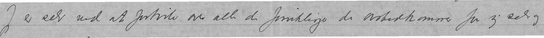Jeg er selv ved at fortvile over alle de forvklinger de avstedkommer for sig selv og
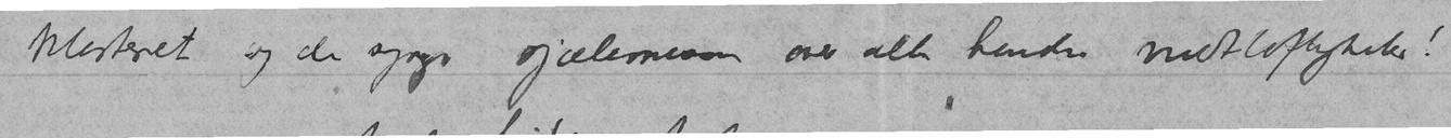klosteret og de synges sjælemessen over alle hendes vidtløftigheter!
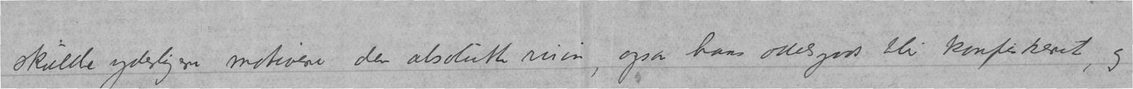skulde yderligere motivere den absolutte ruin, ogsaa hans adelsgods blir konfiskeret, og
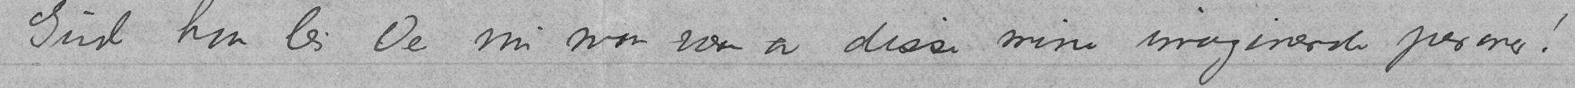Gud hvor lei De nu maa være av disse mine imaginærde personer!
